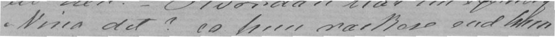Nina det? er hun raskere end hun
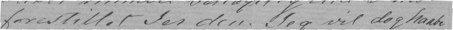forestillet Jer den. Jeg vil dog haabe
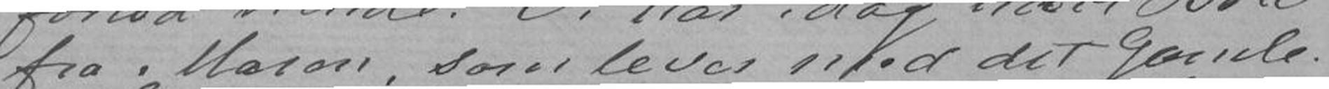fra Maren, som lever med det Gamle.
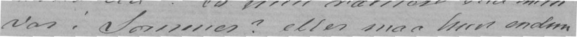var i Sommer? eller maa hun endnu
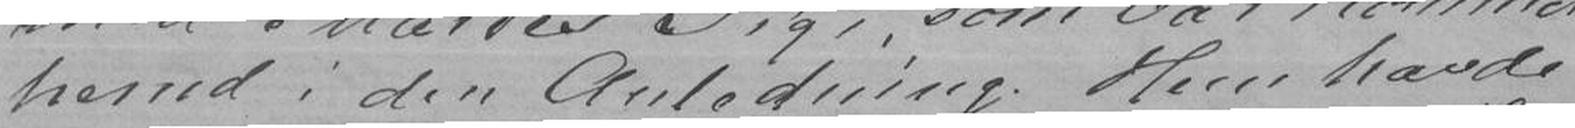herud i den Anledning. Hun havde
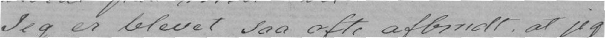Jeg er blevet saa ofte afbrudt. at jeg
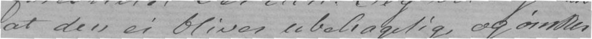at den ei bliver ubehagelig og ønsker
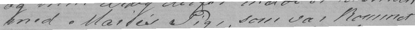med Maries Pige, som var kommet
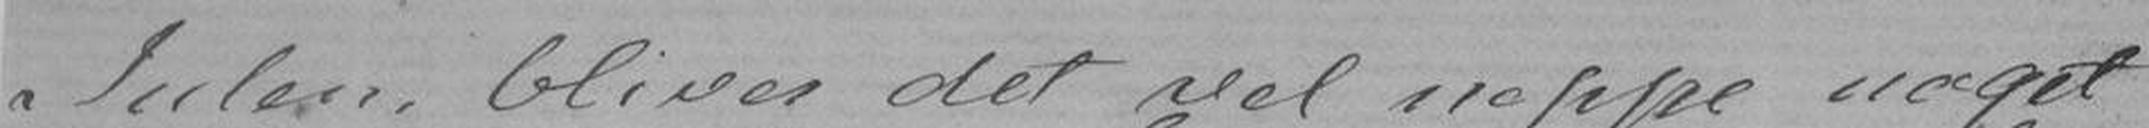Julen bliver det vel neppe noget
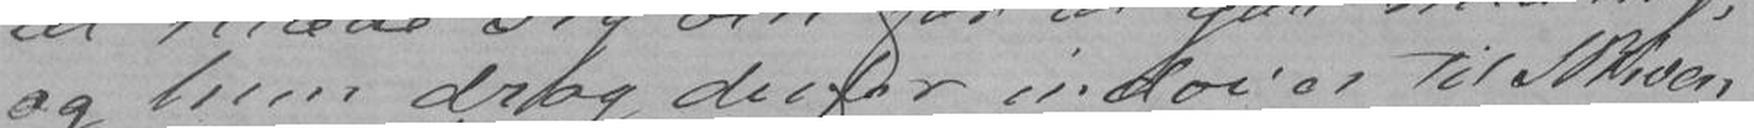og hun drog derfor indover til Skiven
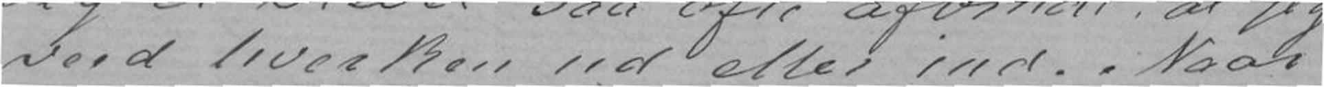veed hverken ud eller ind. Naar
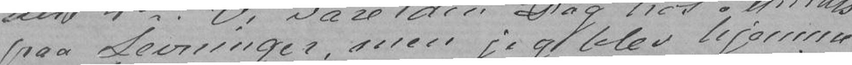paa Levninger, men jeg blev hjemme
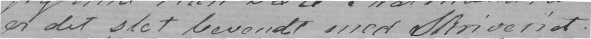er det slet bevendt med skriveriet.
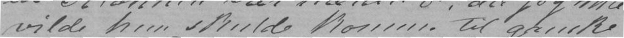vilde hun skulde komme til ganske
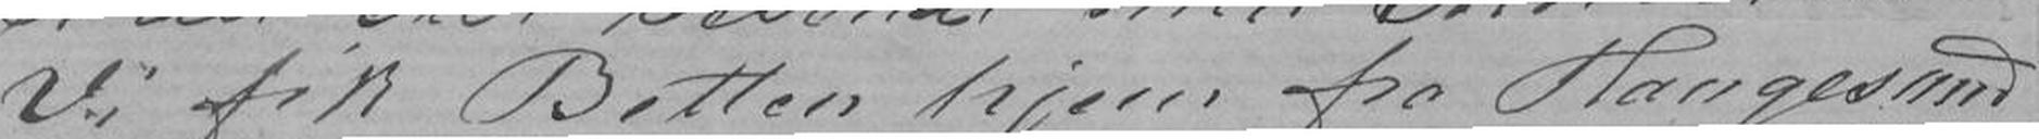Vi fik Betten hjem fra Haugesund
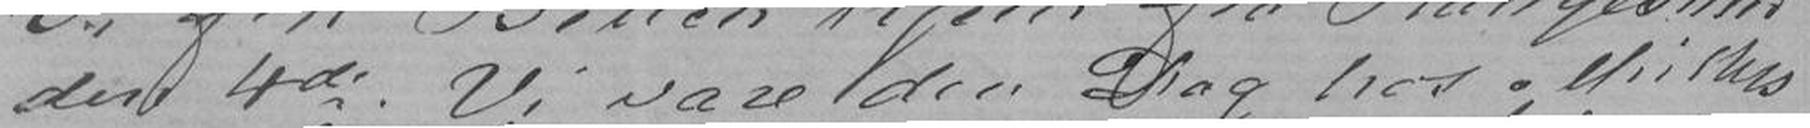den 4de. Vi vare den Dag hos Müller
